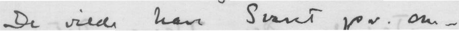De vilde have Svaret på om-
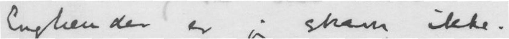Englænder er jeg skam ikke.
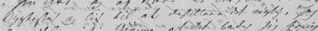Dygtighed og Tid til at destillere det rigtig. Jeg
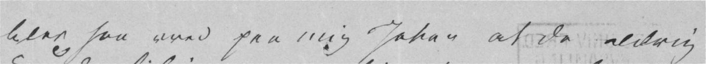blev saa vred paa mig Johan at De aldrig
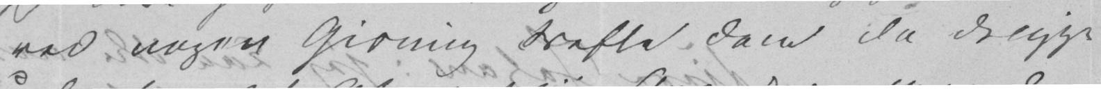ved nogen Gisning treffe dem da de ligge
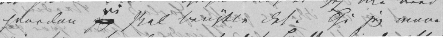hvordan jeg vi skal benytte det. Thi jeg maae
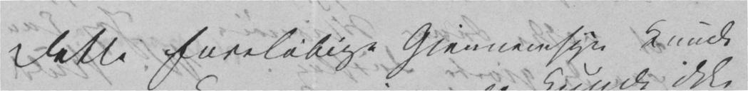Dette foreløbige Gjennemsyn kunde
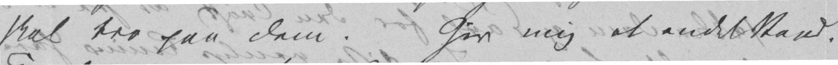skal tro paa dem. Giv mig et andet Raad.
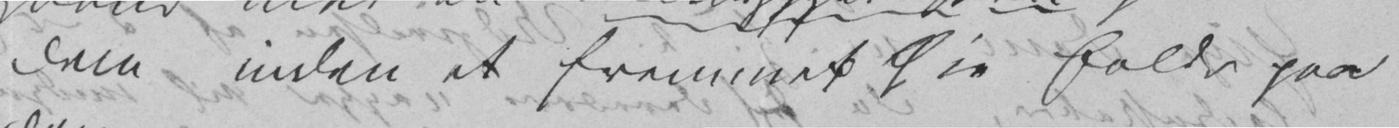dem inden et fremmet Øie falder paa
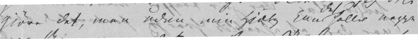gjøre det, men uden min Hjælp kan det  heller neppe
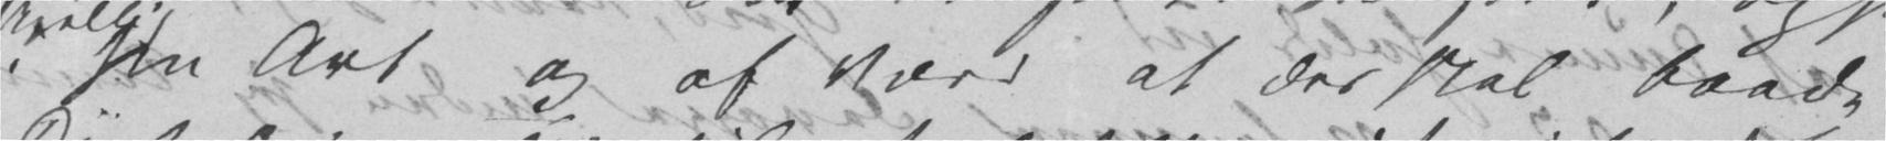i sin Art og af Værd at der skal baade
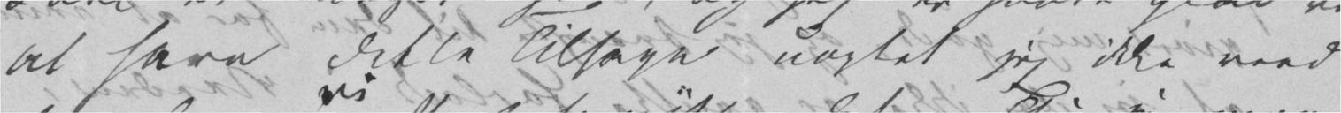at have dette Tilsagn uagtet jeg ikke veed
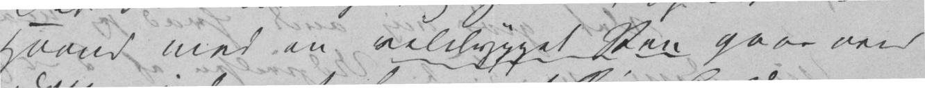Haand med en veldyppet Pen gaae over
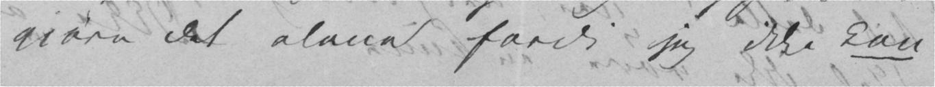gjøre det alene fordi jeg ikke kan
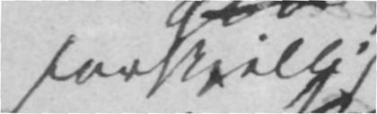forskjellig
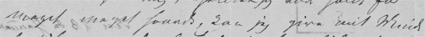meget meget haardt, kan jeg give mit Minde
